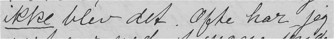ikke blev det. Ofte har jeg
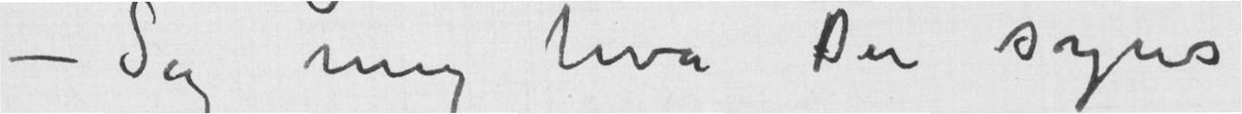– Sig mig hva Du synes
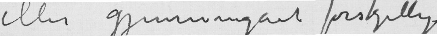eller gjennemgået forskjellige
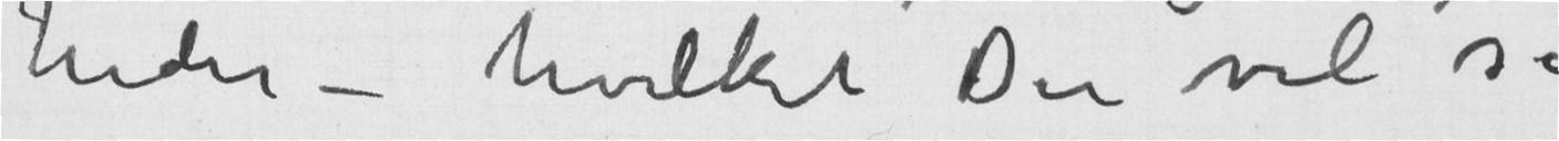heder – hvilket Du vil se
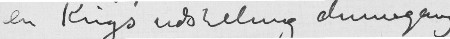en Krigsudstilling dennegang
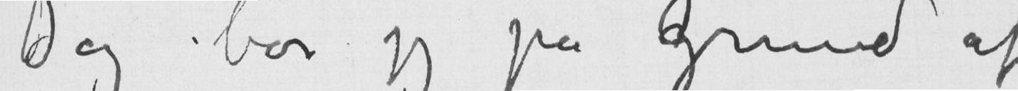Dog bør jeg på Grund af
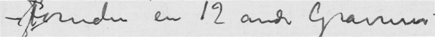– Foruden en 12 andre Gravurer
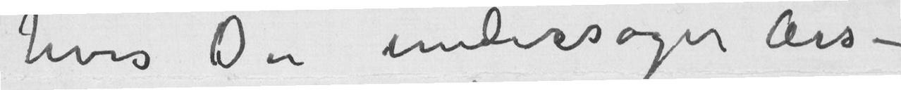hvis Du undersøger Års-
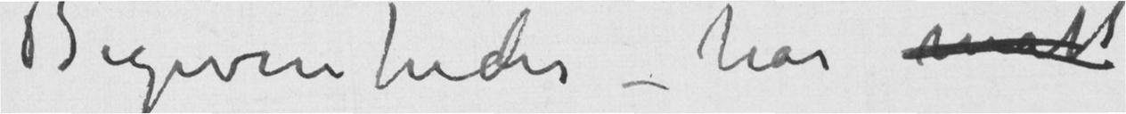Begivenheder – har malt
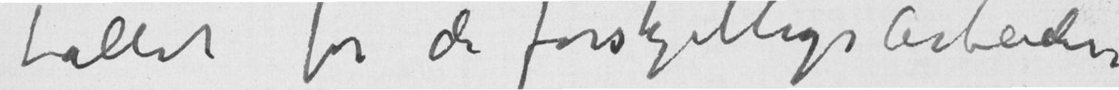tallet for de forskjellige Arbeider
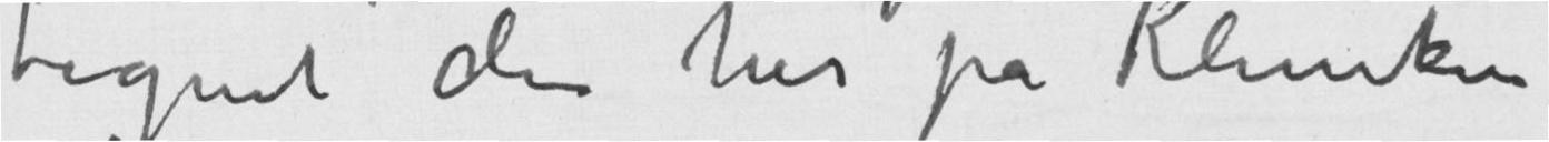tegnet den her på Kliniken
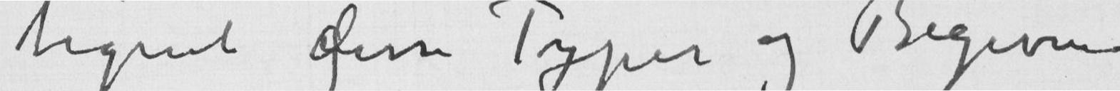tegnet gdisse Typer og Begiven
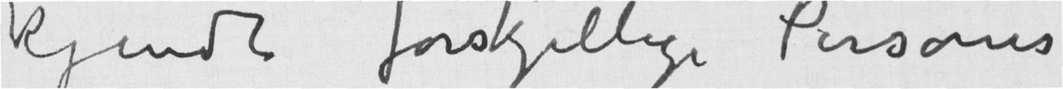kjendt forskjellige Personer
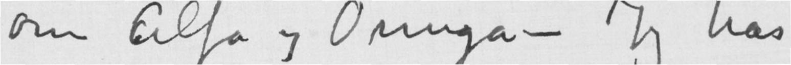om Alfa og Omega – Jeg har
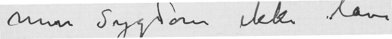min Sygdom ikke lave
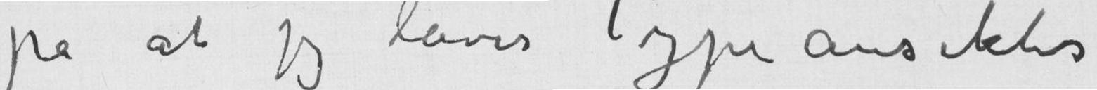på at jeg laver Typeansikter
